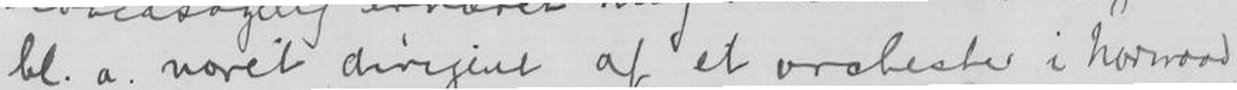bl.a. voret dirigent af et orchester i Norwood
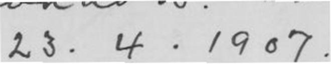23.4.1907
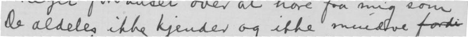De aldeles ikke kjender og ikke mindre fordi
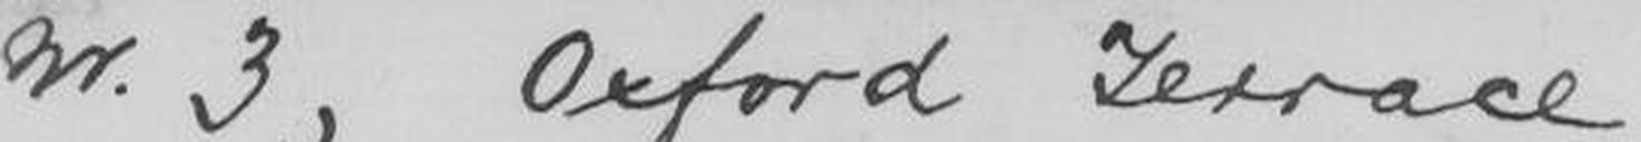Nr 3, Oxford Terrace
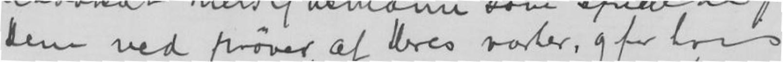Dem ved prøvet af Deres værker, og for hans
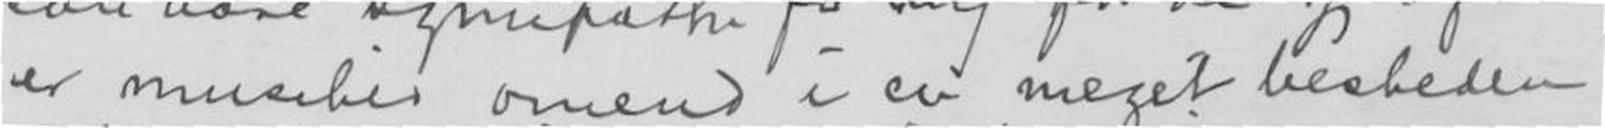er musiker omend i en meget beskeden
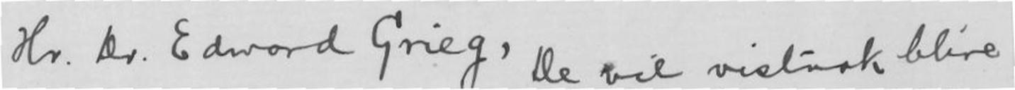Hr. Dr. Edvard Grieg, De vil vistnok blive
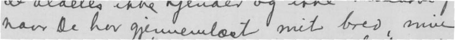naar De her gjennemlæst mit brev, min
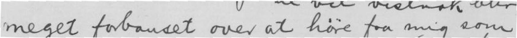meget forbauset over at høre fra mig som
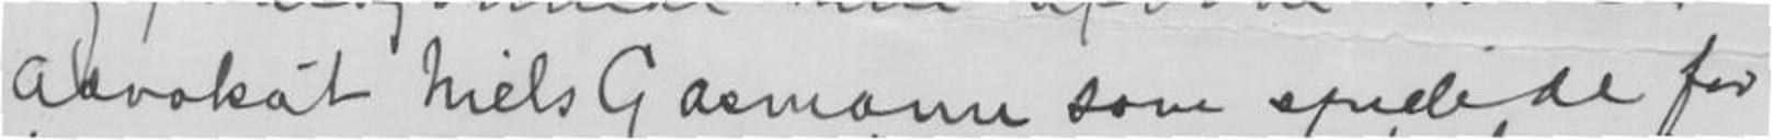Advokat Niel Gasmann som spillede for
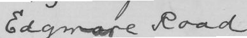Edgmare Road
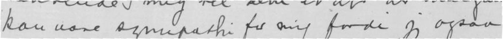kan Være sympathi for mig fordi jeg ogsaa
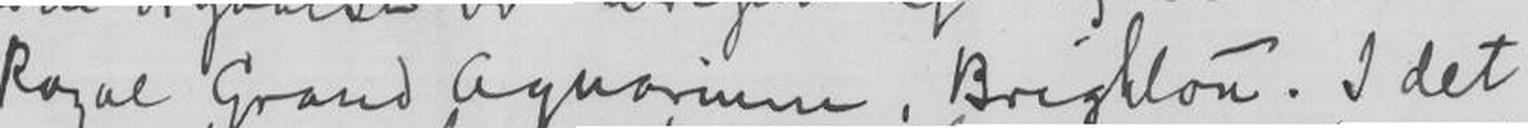Royal Grand Aquarium. Brighton. I det
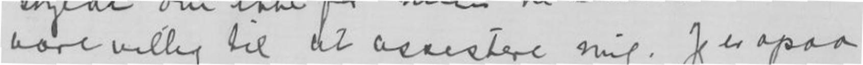være villig til at assetere mig. Jeg er ogsaa
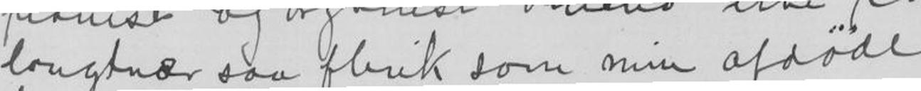langtnær saa flink som min afdøde
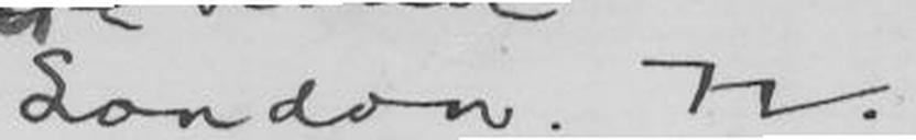London N.
